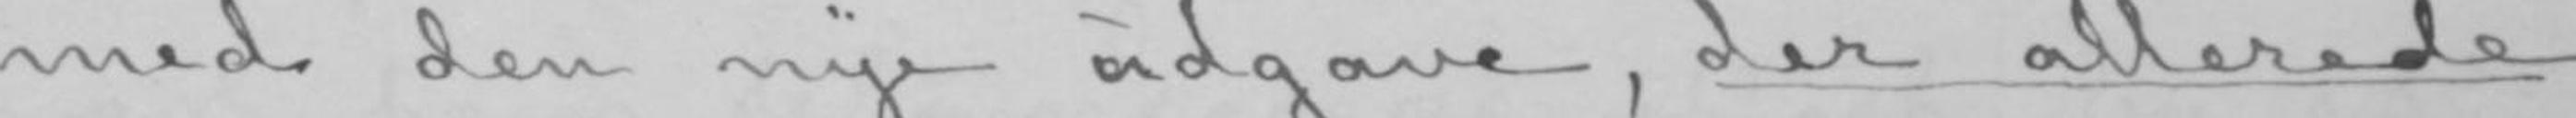med den nye udgave, der allerede
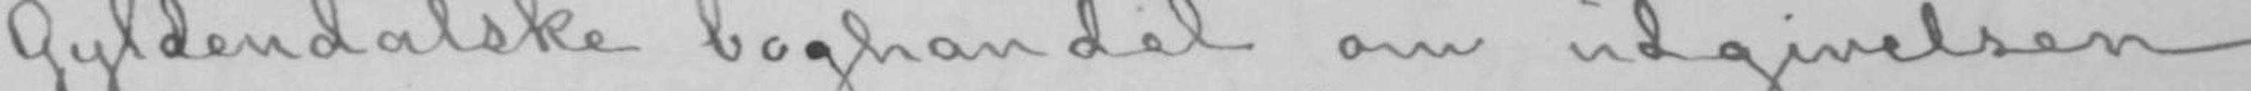Gyldendalske boghandel om udgivelsen
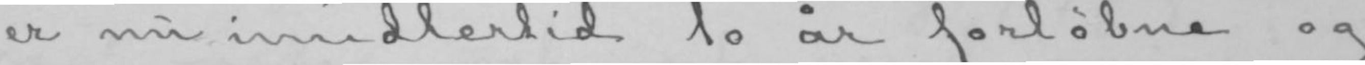er nu imidlertid to år forløbne og
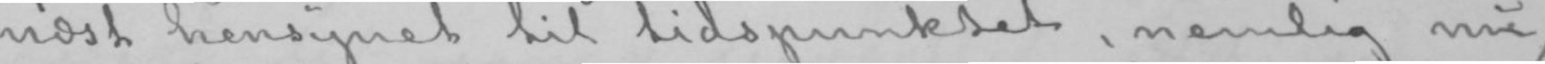næst hensynet til tidspunktet, nemlig nu,
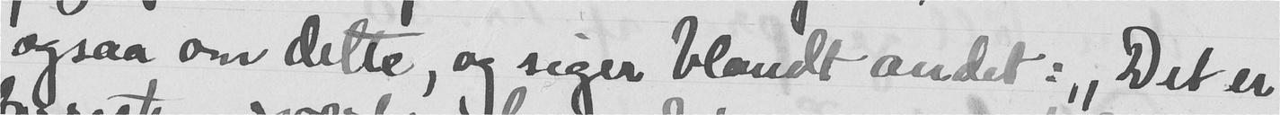ogsaa om dette, og siger blandt andet: "Det er
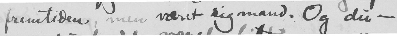fremtiden, men været rig mand. Og du -
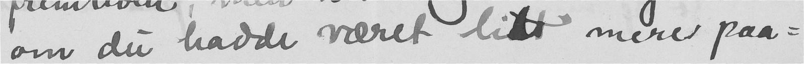om du havde været lidt mere paa-
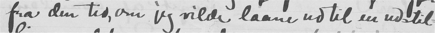fra den tid, om jeg vilde laane ud til en udstil-
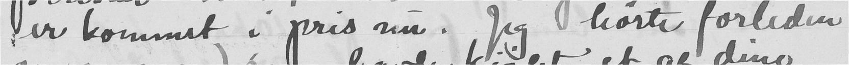er kommet i pris nu. Jeg hørte forleden
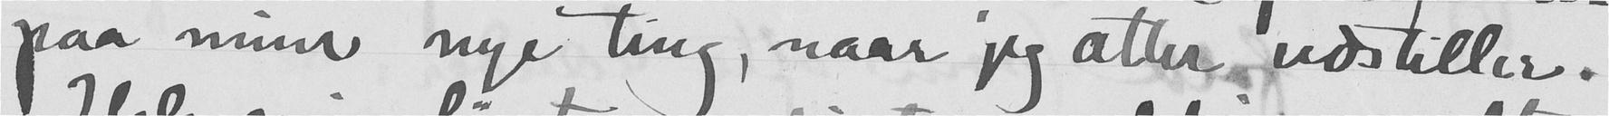paa mine nye ting, naar jeg atter udstiller.
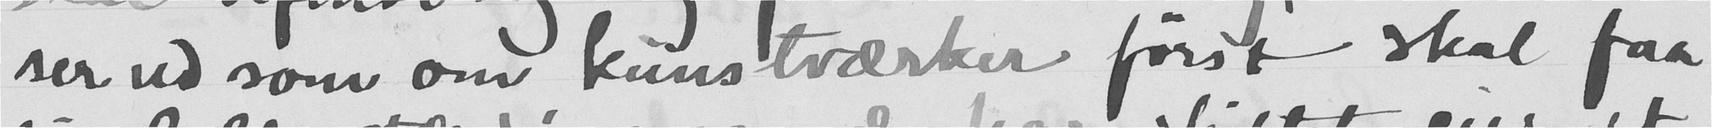ser ud som om kunstværker først skal faa
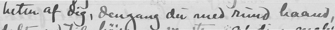heten af dig, dengang du med rund haand,
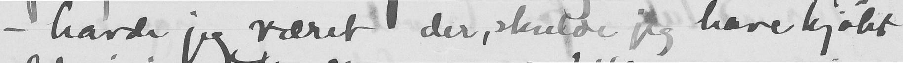- havde jeg været der, skulde jeg have kjøbt
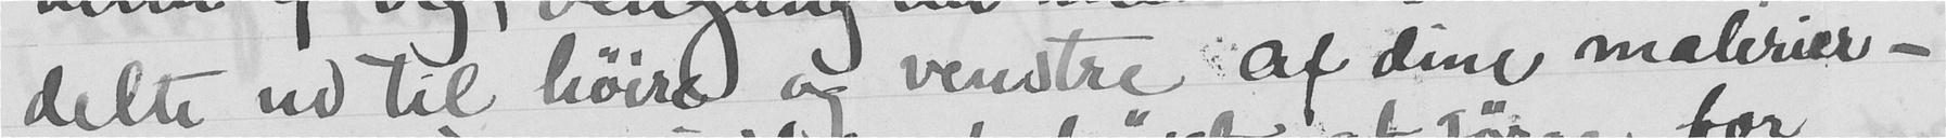delte ud til høire og venstre af dine malerier -
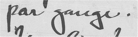par gange.
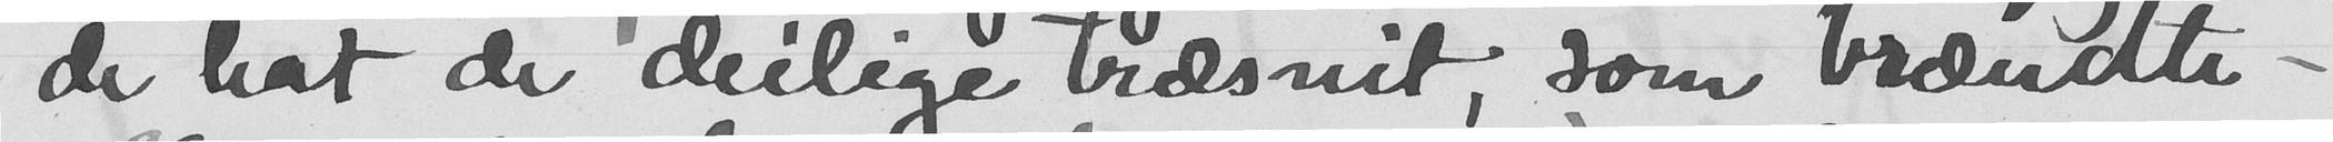de hat de deilige træsnit, som brændte -
